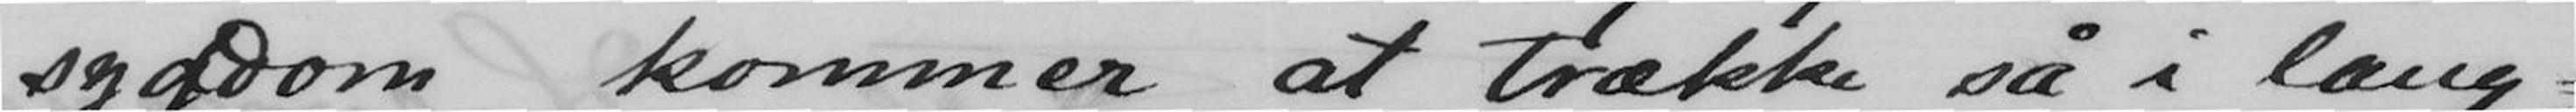sygdom kommer at trække så i lang-
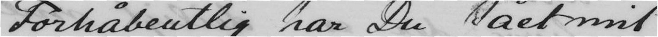Forhåbentlig har Du fået mit
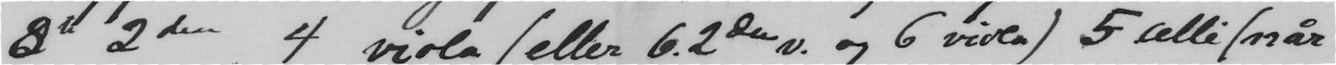8te 2den, 4 viola(eller 6. 2denv. og 6 viola) 5 celli (når
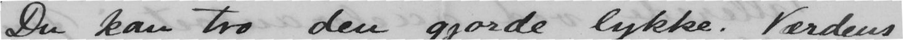Du kan tro den gjorde lykke. Værdens
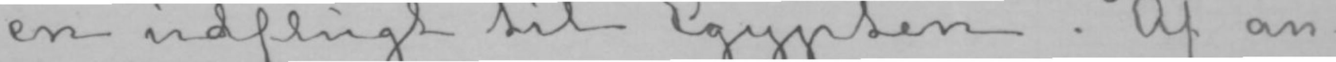en udflugt til Egypten. Af an-
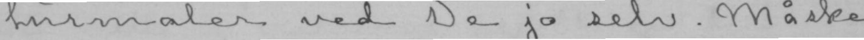turmaler ved De jo selv. Måske
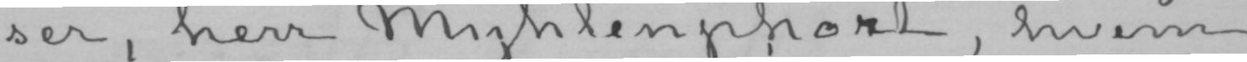ser, herr Myhlenphort, hvem
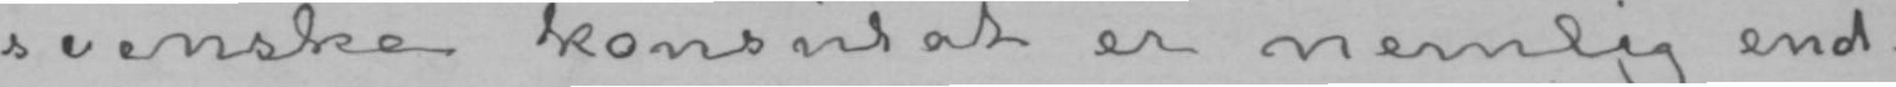svenske konsulat er nemlig end-
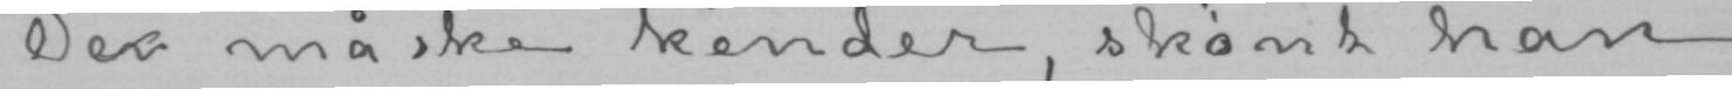Der måske kender, skønt han
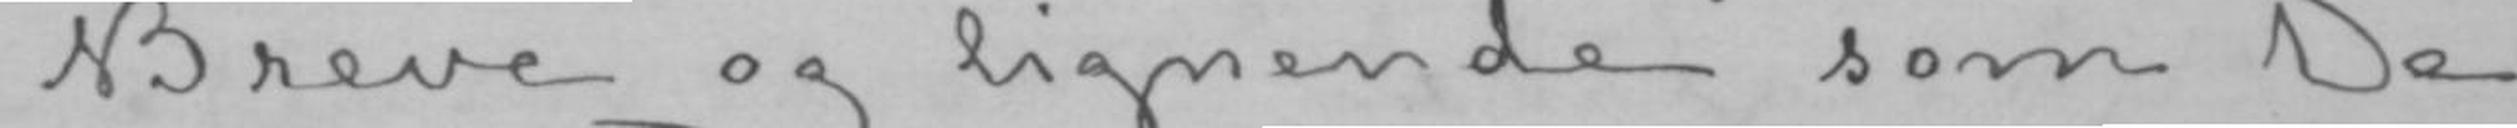Breve og lignende som De
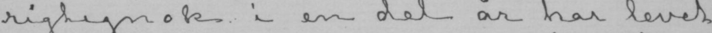rigtignok i en del år har levet
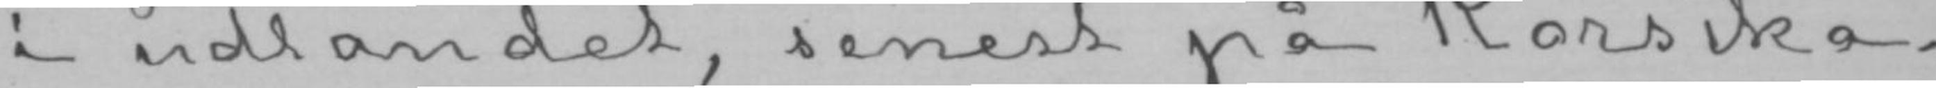i udlandet, senest på Korsika.
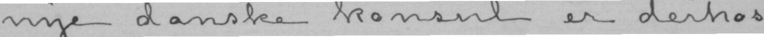nye danske konsul er derhos
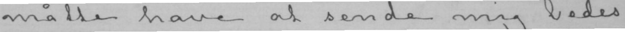måtte have at sende mig bedes
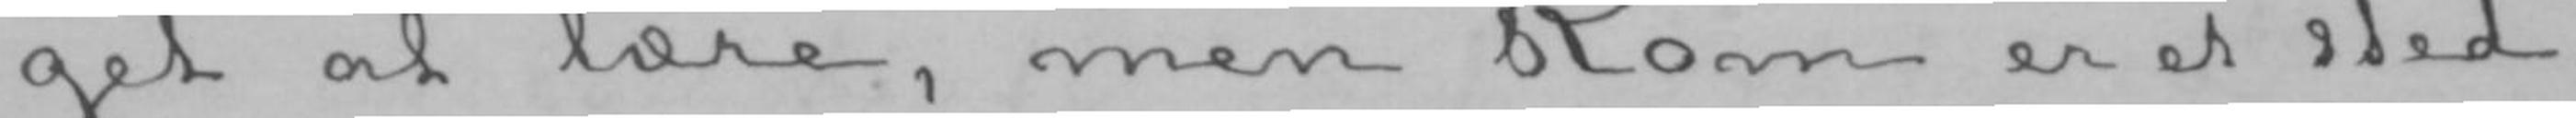get at lære, men Rom er et sted,
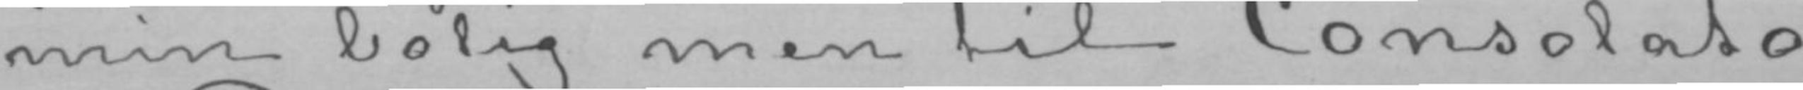min bolig men til Consolato
